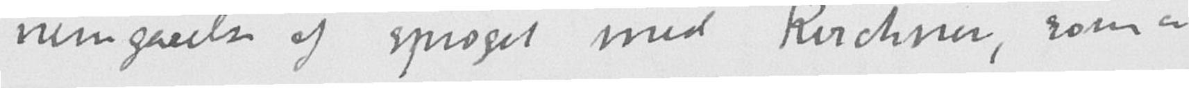nemgaaelse af sproget med Kirchner, som er
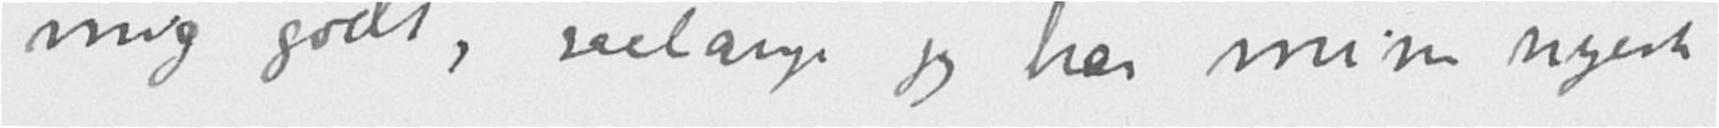mig godt, saalænge jeg har mine nyeste
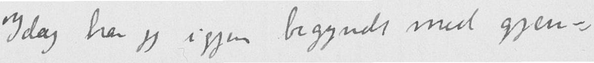Idag har jeg igjen begyndt med gjen-
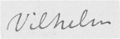Vilhelm
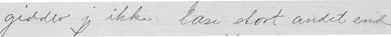gidder jeg ikke læse stort andet end
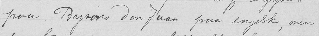paa Byrons Don Juan paa engelsk, men
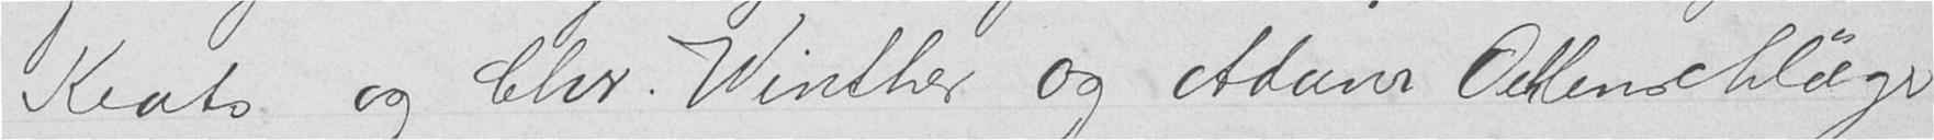Keats og Chr. Winther og Adam Oehlenschläger
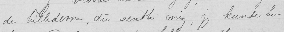de billederne, du sendte mig, jeg kunde be-
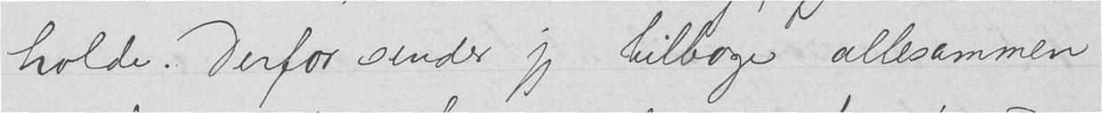holde. Derfor sender jeg tilbage allesammen
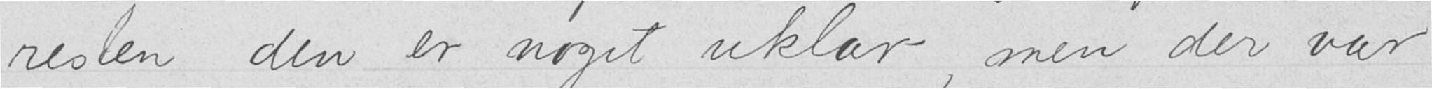resten den er noget uklar, men der var
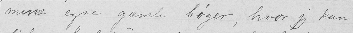mine egne gamle bøger, hvor jeg kan
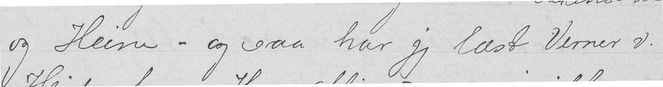og Heine - og saa har jeg læst Verner v.
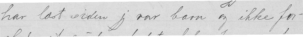har læst siden jeg var barn og ikke for-
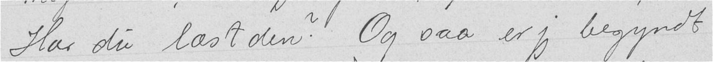Har du læst den? Og saa er jeg begyndt
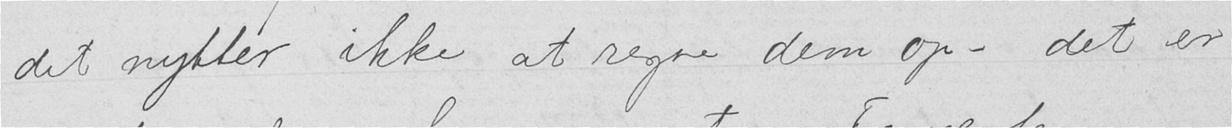det nytter ikke at regne dem op - det er
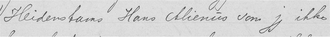Heidenstams "Hans Alienus" som jeg ikke
